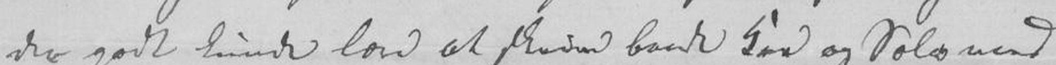da godt kunde lære at skrive baade Kor og Solo mest
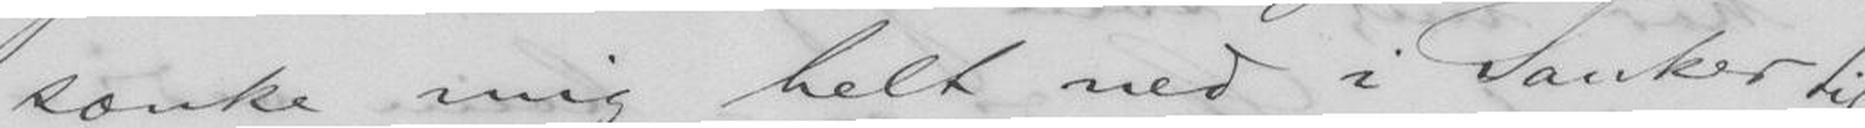sænke mig helt ned i Tanker til
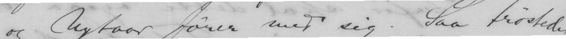og Nytaar fører med sig. Saa trøstede
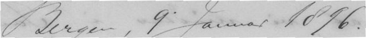Bergen, 9 Januar 1896.
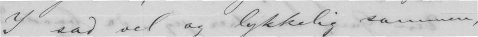I sad vel og lykkelig sammen,
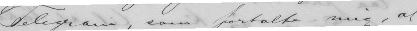Telegram, som fortalte mig, at
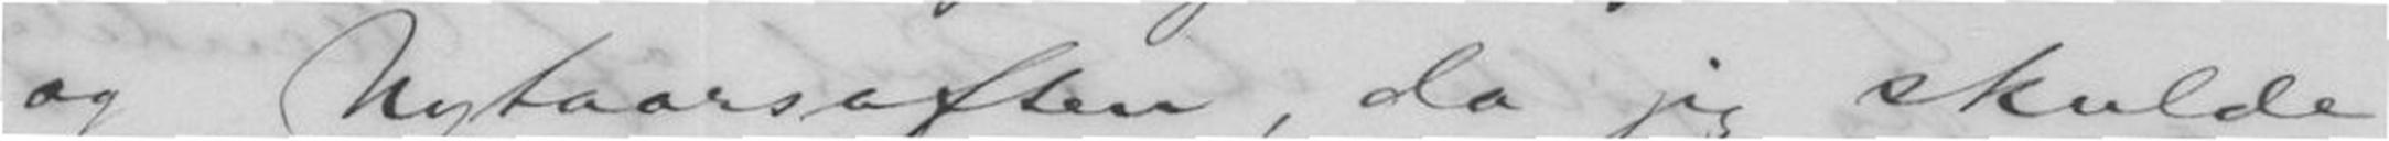og Nytaarsaften, da jeg skulde
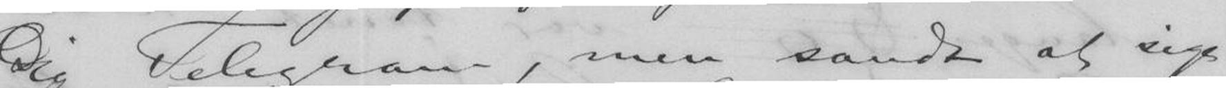Dig Telegram, men sandt at sige
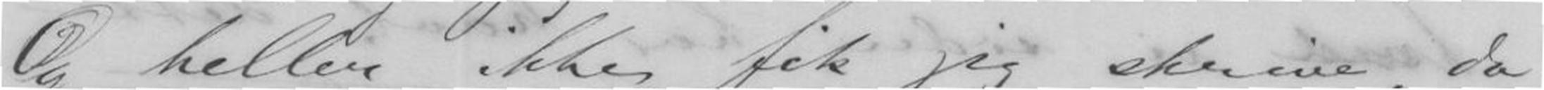Og heller ikke fik jeg skrive, da
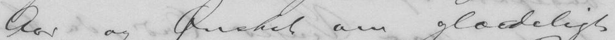Aar og Ønsket om glædeligt
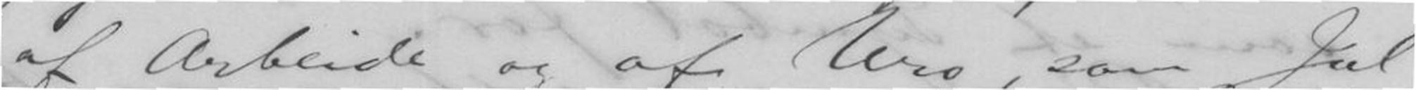af Arbeidet og af Uro, som Jul
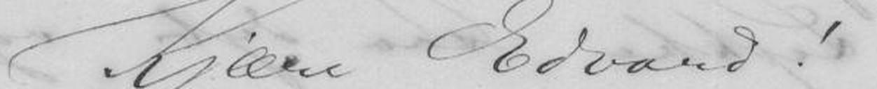Kjære Edvard!
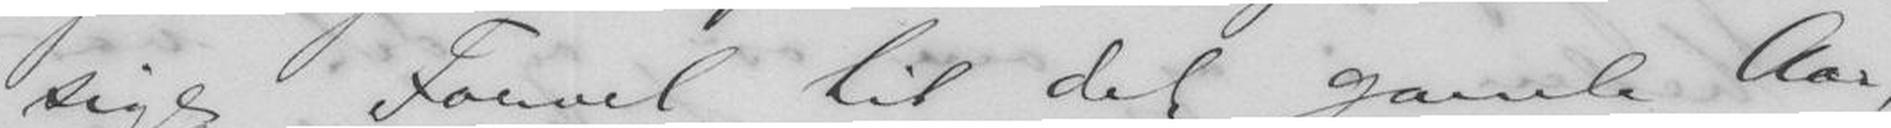sige Farvel til det gamle Aar,
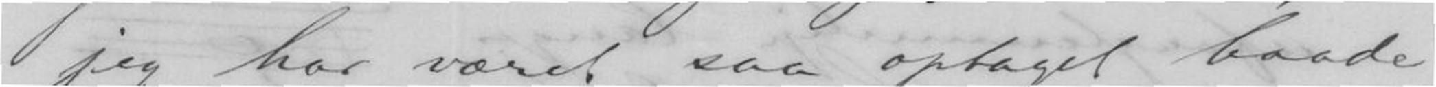jeg har været saa optaget baade
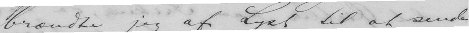brændte jeg af Lyst til at sende
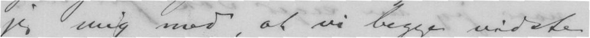jeg mig med, at vi begge vidste,
